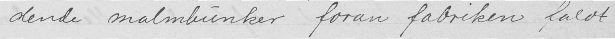dende malmbunker foran fabriken faldt
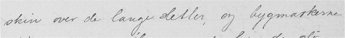skin over de lange sletter, og bygmarkerne
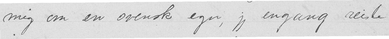mig om en svensk egn, jeg engang reiste
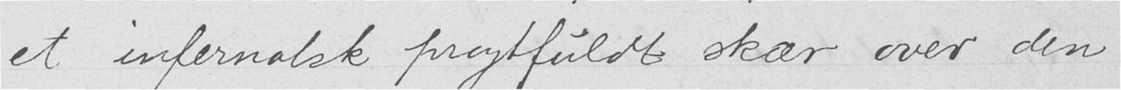et infernalsk pragtfuldt skær over den
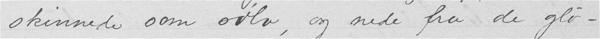skinnede som sølv, og nede fra de glø-
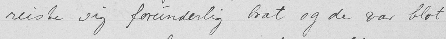reiste sig forunderlig brat og de var blot
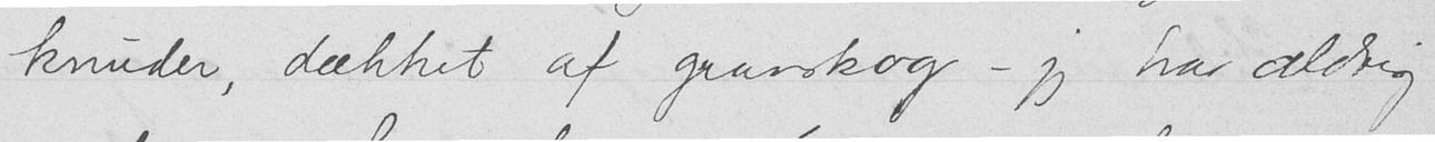knuder, dækket af granskog — jeg har aldrig
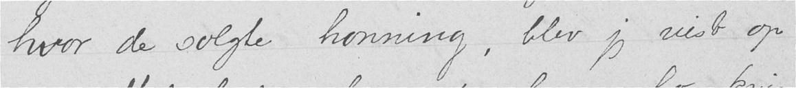hvor de solgte honning, blev jeg vist op
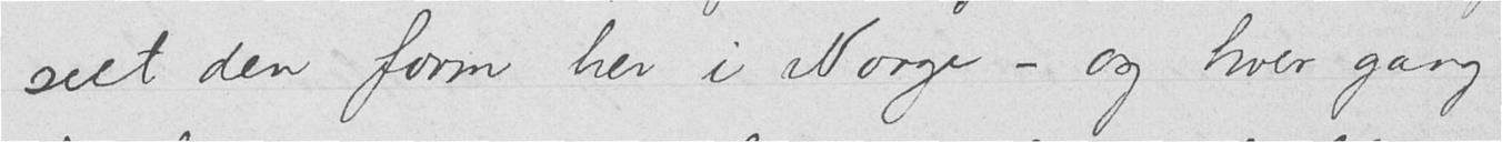seet den form her i Norge — og hver gang
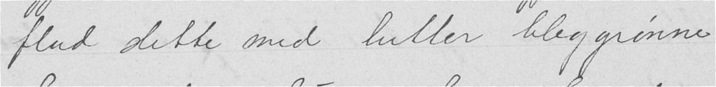flad slette med lutter bleggrønne
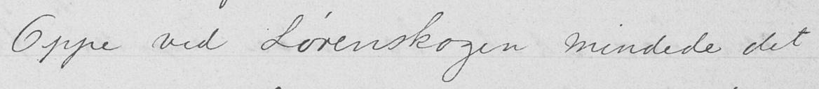Oppe ved Lørenskogen mindede det
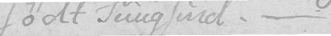sødt Tungsind. –
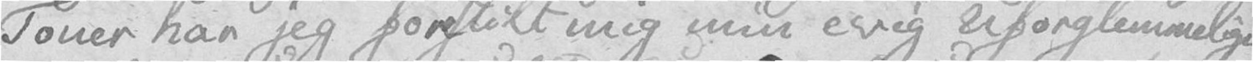Toner har jeg forestilt mig min evig Uforglemmelige
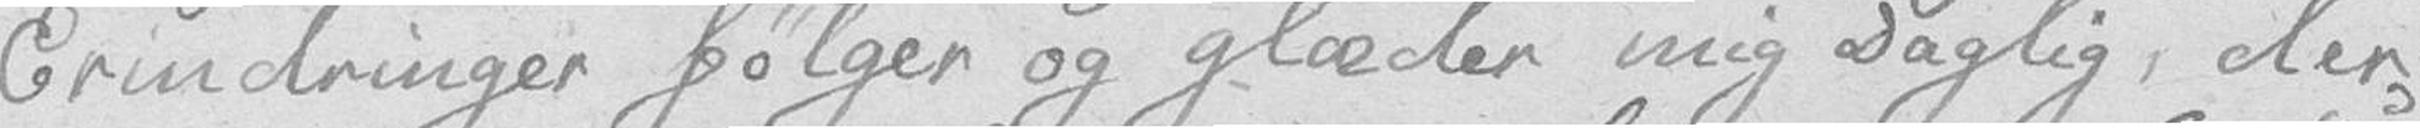Erindringer følger og glæder mig Daglig, der
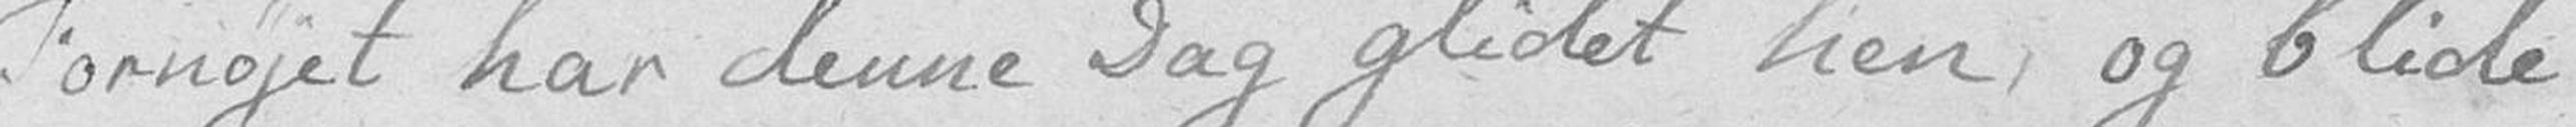Fornøjet har denne Dag glidet hen, og blide
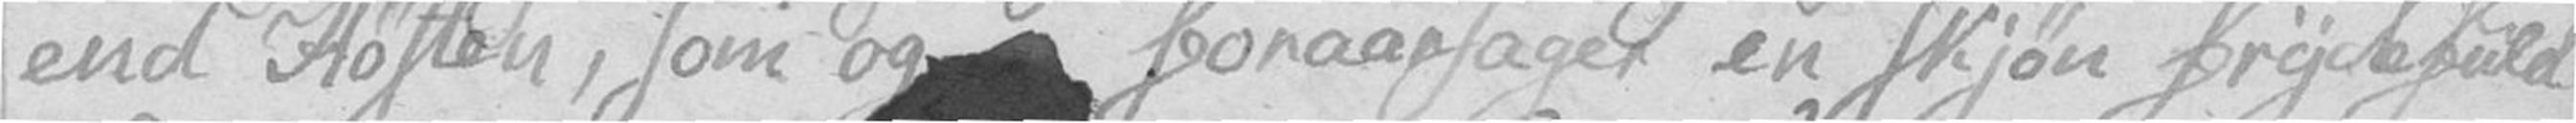end Høsten, som og foraarsaget en skjøn frydefuld
